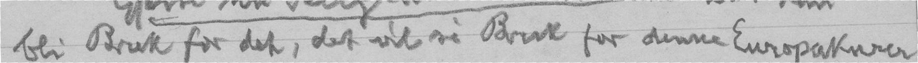bli Bruk for det, det vil si Bruk for denne Europakurer
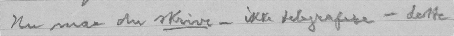Nu maa du skrive - ikke telegrafere - dette
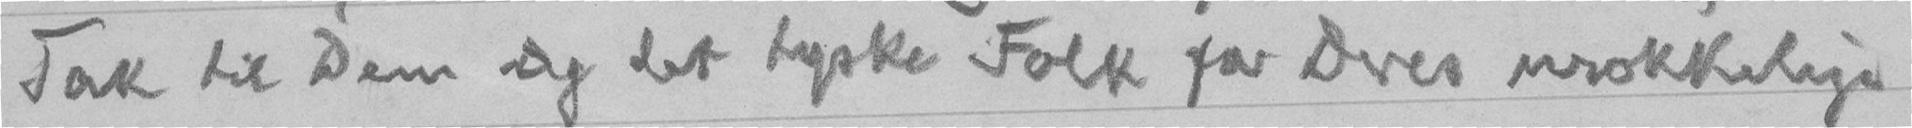Tak til Dem og det tyske Folk for Deres urokkelige
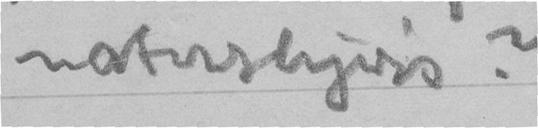naturligvis?
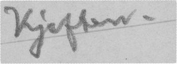Kjeften.
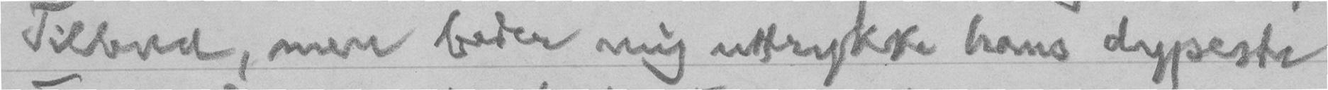Tilbud, men beder mig uttrykke hans dypeste
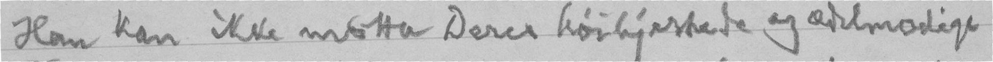Han kan ikke motta Deres høihjertede og ædelmodige
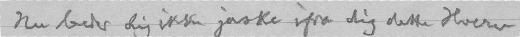Nu beder dig ikke jaske ifra dig dette Hverv
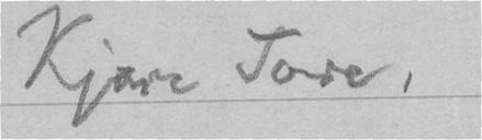Kjære Tore,
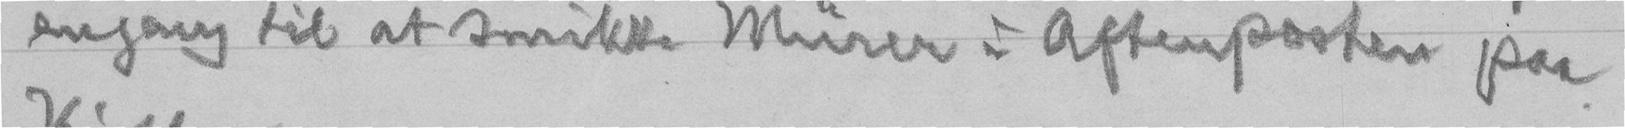engang til at smikke Mürer i Aftenposten paa
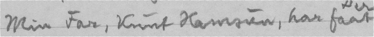Min Far, Knut Hamsun, har faat
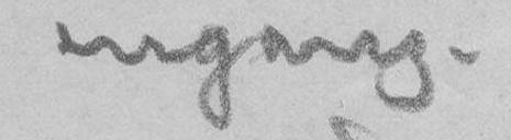engang.
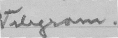Telegram.
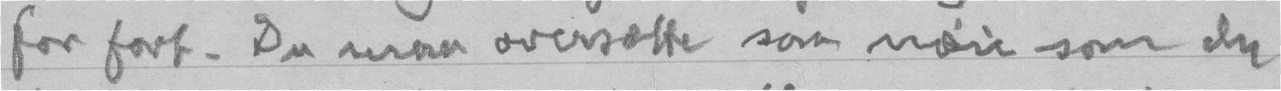for fort. Du maa oversætte saa nøie som du
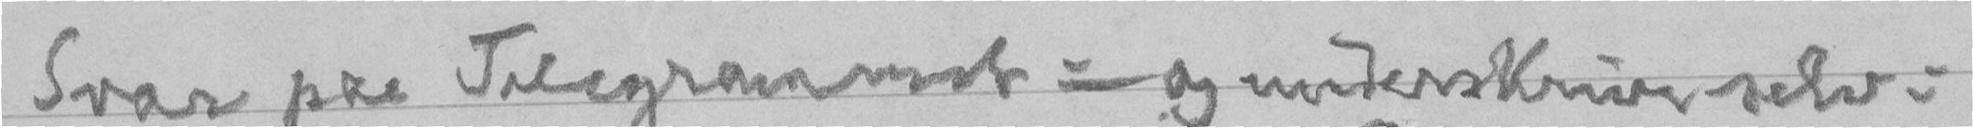Svar paa Telegrammet - og underskrive selv:
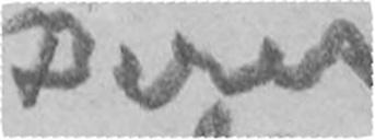Deres
